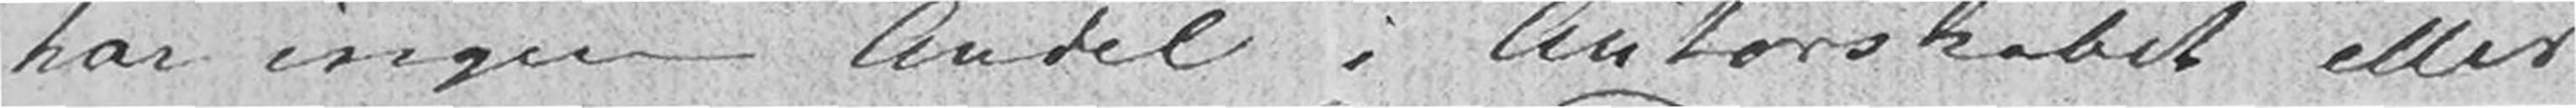har ingen Andel i Autorskabet eller
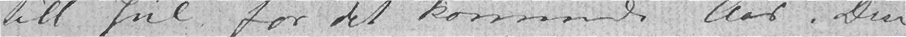till Jul for det kommende Aar. Din
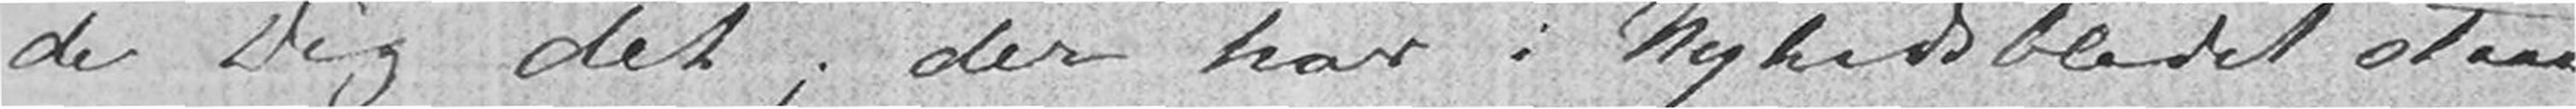de Dig det; der har i Nyhedsbladet staaet
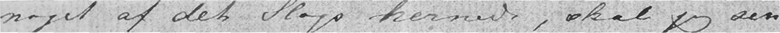noget af det Slags hernede, skal jeg sen-
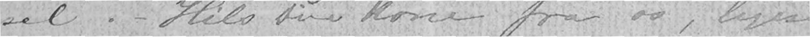sel.- Hils din Kone fra os, ligesaa
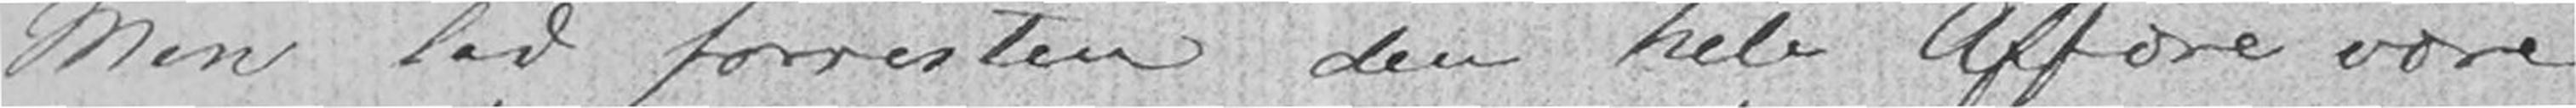Men lad forresten den hele Affære være
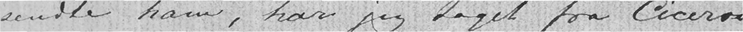sendte ham, har jeg taget fra Ciceros
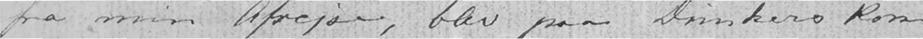fra min Afrejse, blev paa Dunkers Kon-
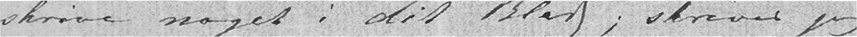skrive noget i dit Blad; skriver jeg
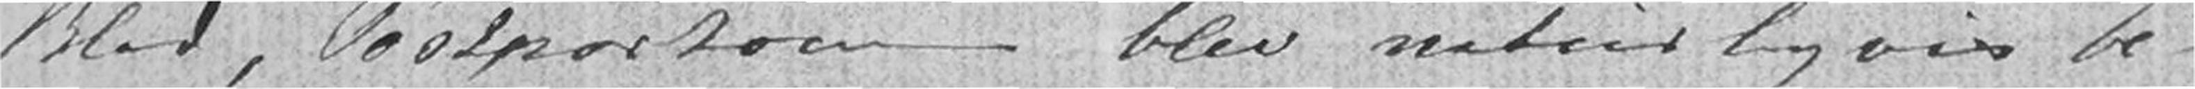Blad, Postportoen blev naturligvis be-
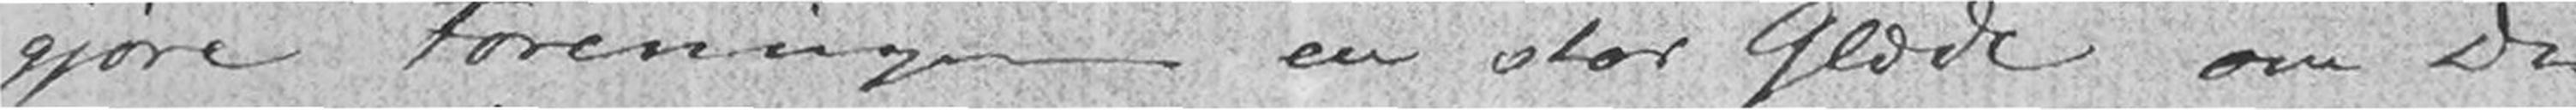gjøre Foreningen en stor Glæde om Du
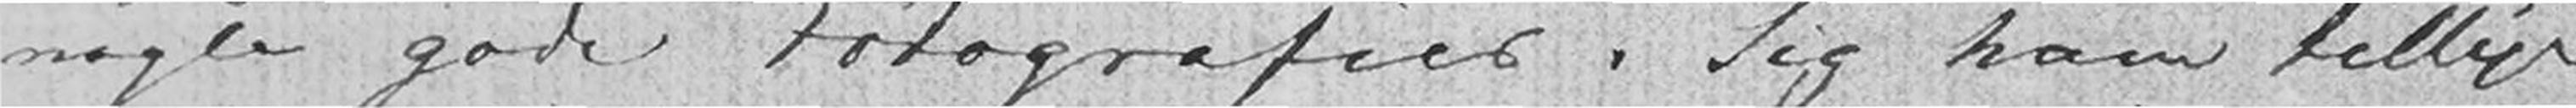nogle gode Fotografier. Sig ham tillige
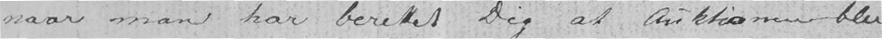naar man har berettet Dig at Auktionen blev
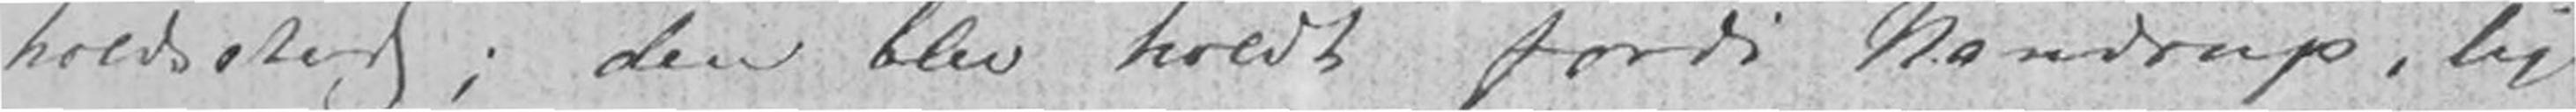holdssted; den blev holdt fordi Nandrup, lige
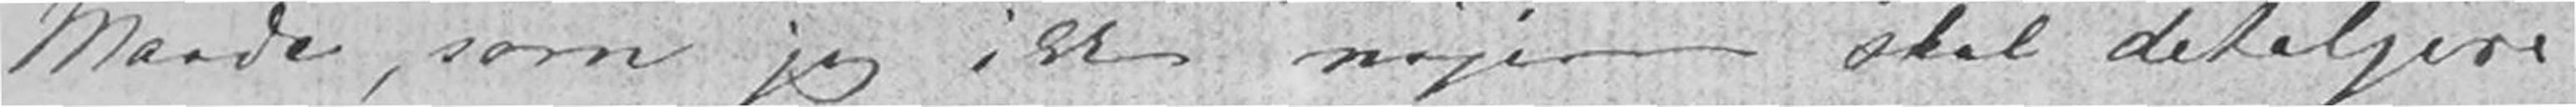Maade, som jeg ikke nøjere skal detaljere.
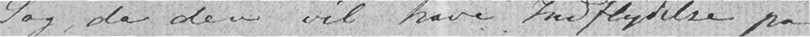Sag, da den vil have Indflydelse paa
